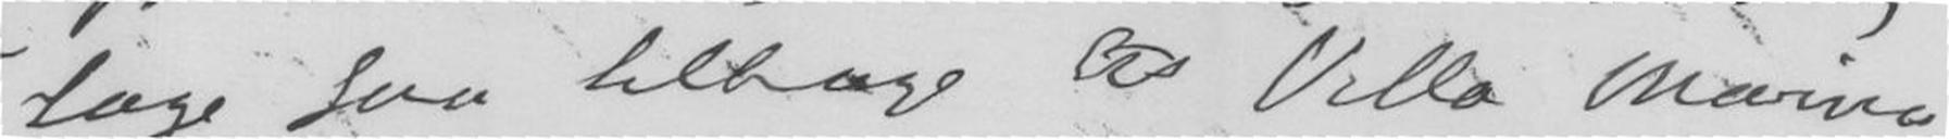tage saa tilbage til Villa Marina
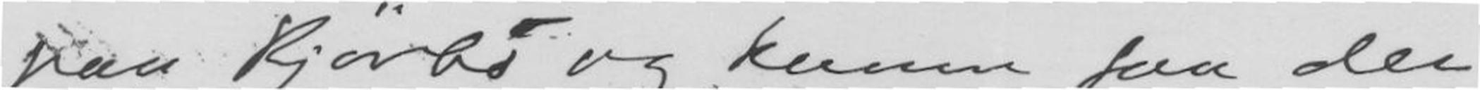paa Kjørbo og kommer saa den
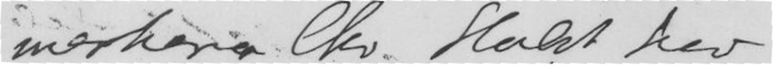merherre Chr. Holst har
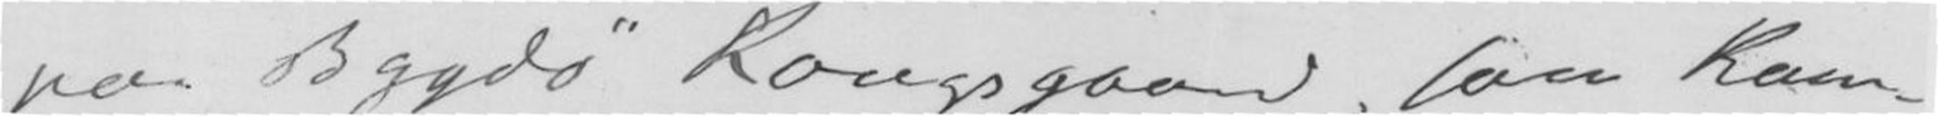paa Bygdø Kongsgaard, som Kam-
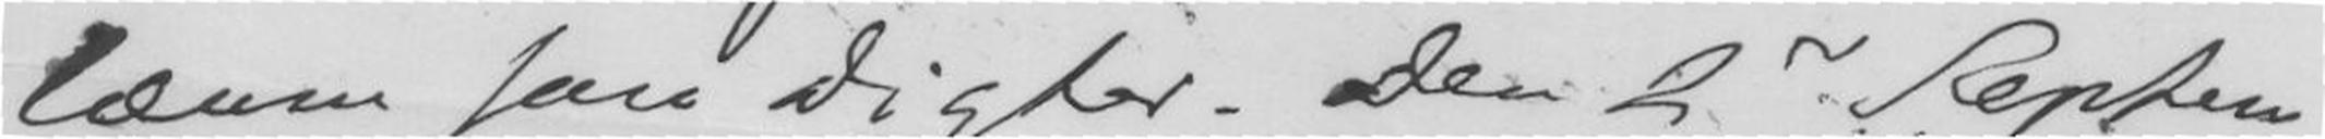læum som Digter - den 2n Septem-
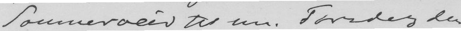Sommerveir til nu. Torsdag den
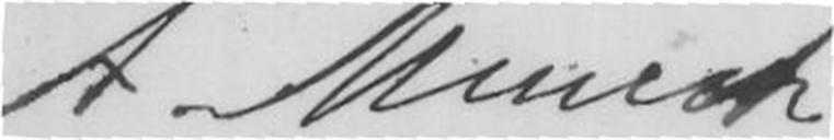A. Munch.
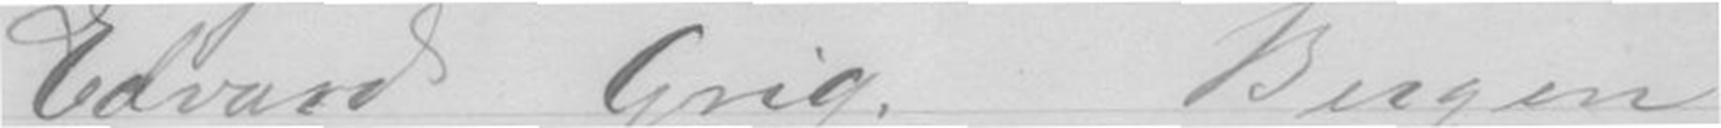Edvard Grieg. Bergen
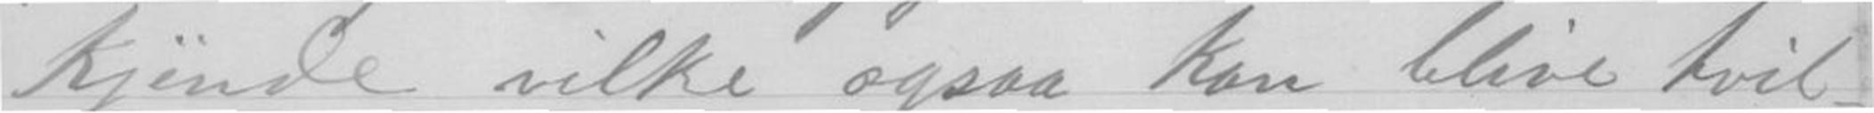kjende vilke ogsaa kan blive tvil-
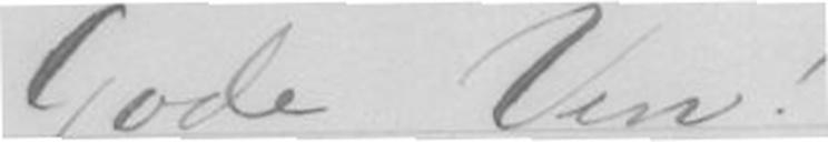Gode Ven!
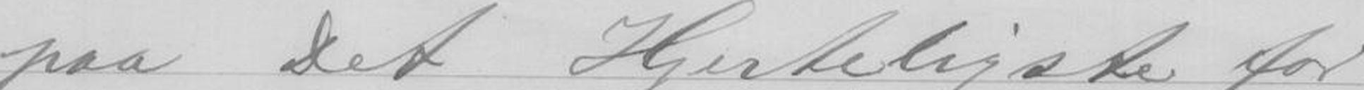paa det hjerteligste for
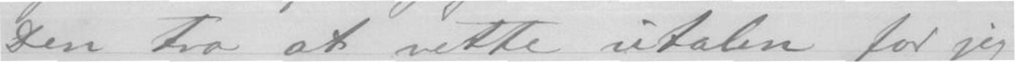den tro at rette utalen for jeg
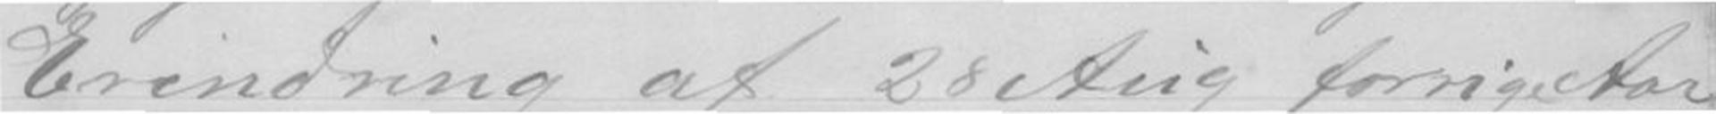Erindring af 28 Aug forrige Aar
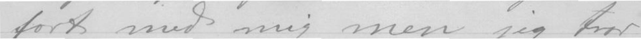fort med mig men jeg tror
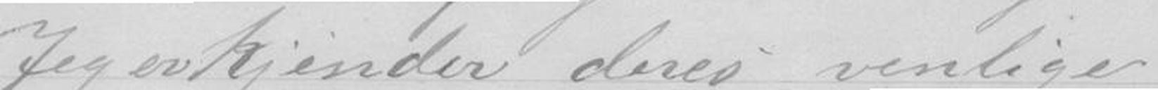Jeg erkjender deres venlige
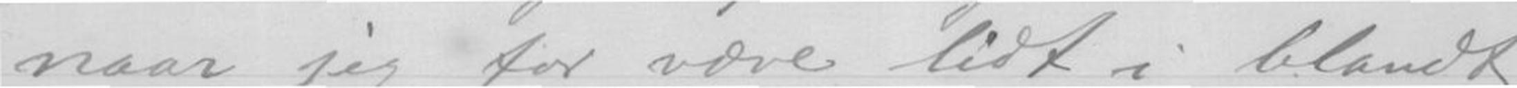naar jeg for være lidt i blandt
